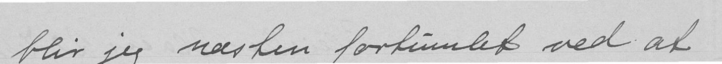blir jeg næsten fortumlet ved at
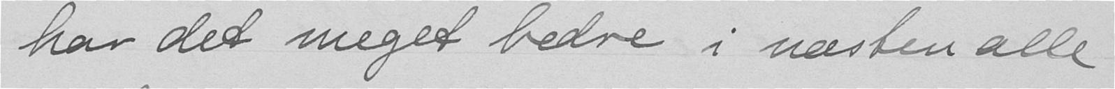har det meget bedre i næsten alle
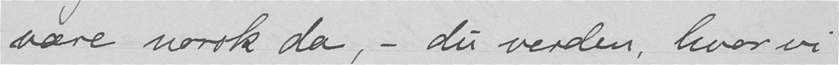være norsk da, - du verden, hvor vi
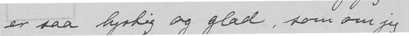er saa lystig og glad, som om jeg
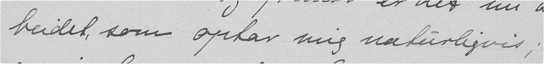beidet, som optar mig naturligvis
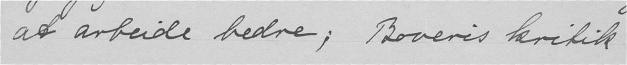at arbeide bedre; Boveris kritik
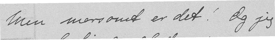Men morsomt er det. Og jeg
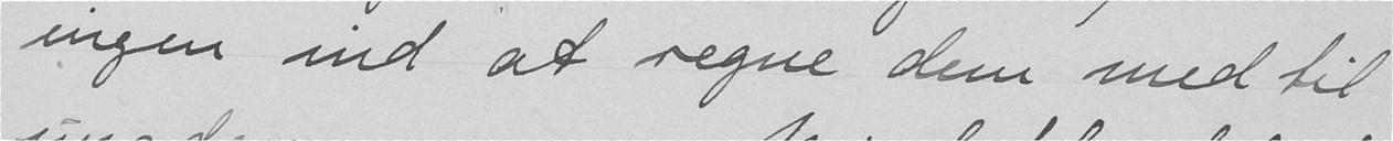ingen ind at regne dem med til
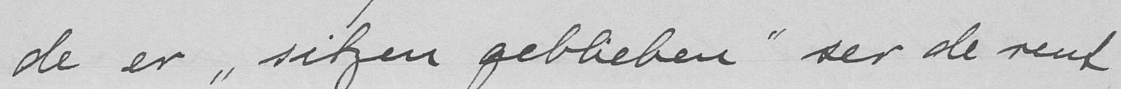de er "sitzen geblieben" ser de rent
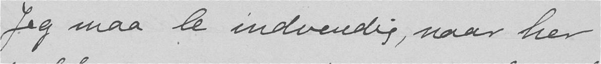Jeg maa le indvendig, naar her
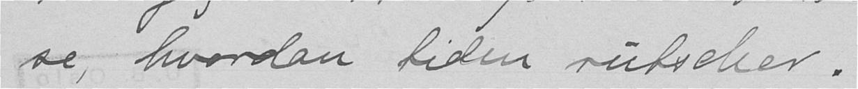se, hvordan tiden rutscher.
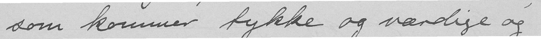som kommer tykke og værdige og
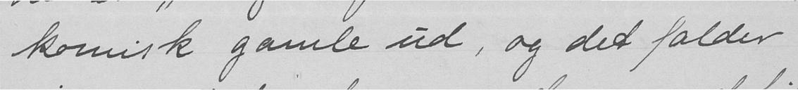komisk gamle ud, og det falder
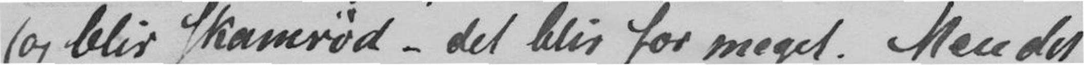og blir skamrød - det blir for meget. Men det
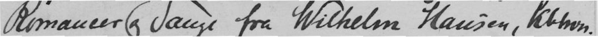Romancer og Sange fra Wilhelm Hansen, Kbhvn.
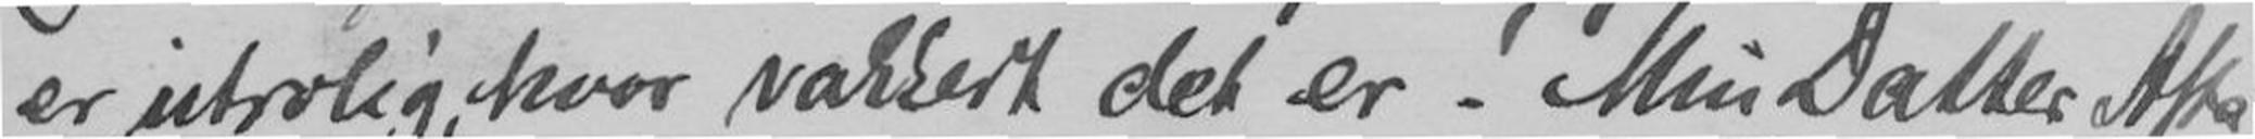er utrolig, hvor vakkert det er! Min Datter Asta
